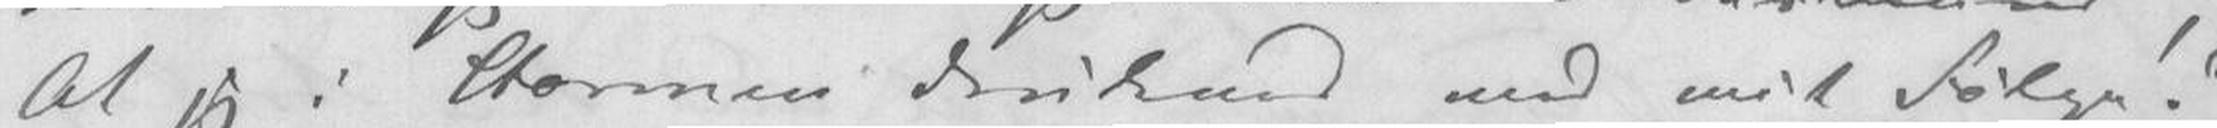At jeg i Stormen druknet med mit Følge?
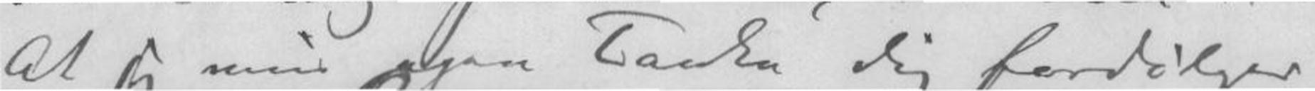At jeg min egen Tanke dig fordølger;
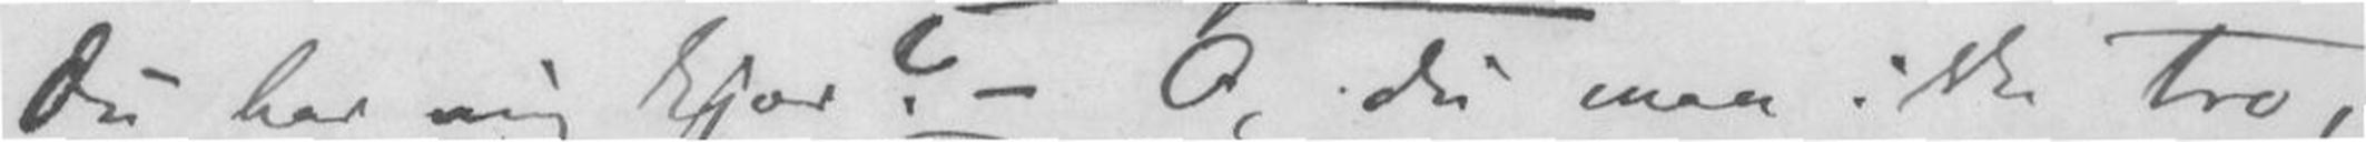Du har mig kjær? - O, du maa ikke tro,
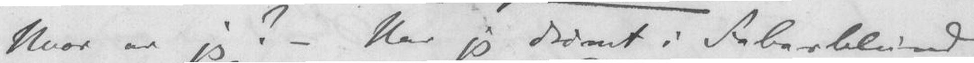Hvor er jeg? - Har jo drømt i Feberblund,
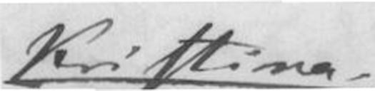Kristina.
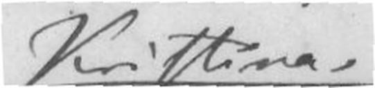Kristina
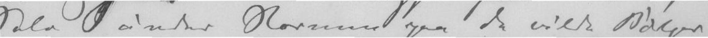Selv under Stormen paa de vilde Bølger.
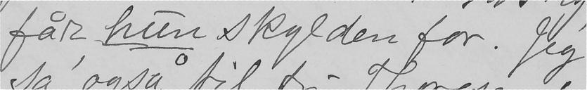får hun skylden for. Jeg
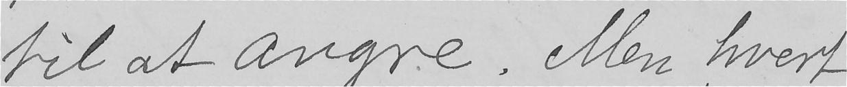til at angre. Men hvert
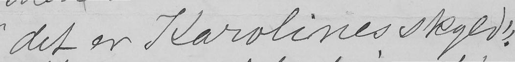det er Karolines skyld".
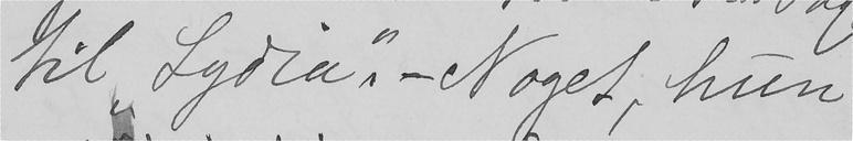til "Lydia". - Noget, hun
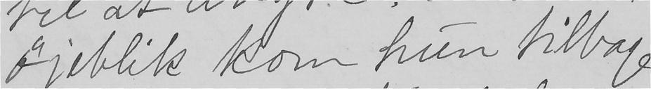øjeblik kom hun tilbage
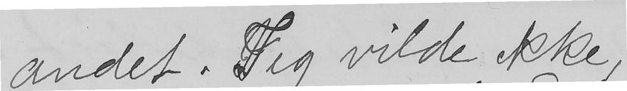andet. Jeg vilde ikke,
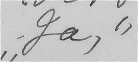"Ja,"
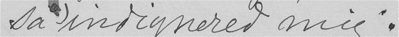sa indignered mig:
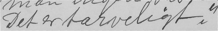Det er tarveligt,"
